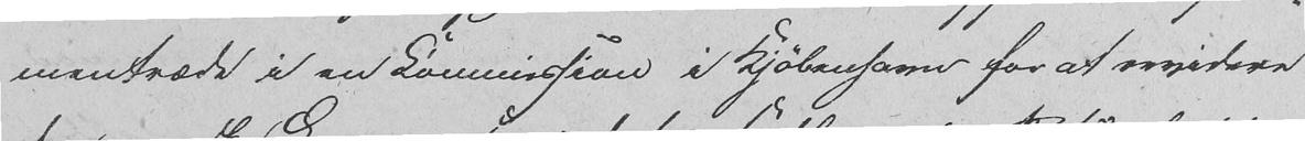mentræde i en Kommission i Kjøbenhavn for at revidere
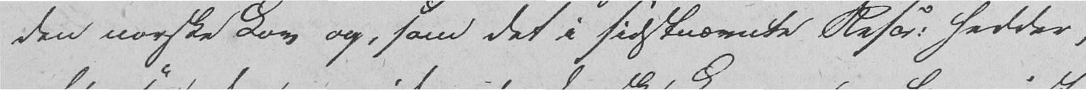den norske Lov og, som det i sidstnævnte Rescr. hedder,
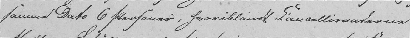samme Dato 6 Personer, hvoriblandt Kancelliraaderne
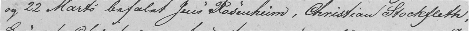og 22 Marts befalet Jens Rosenheim, Christian Stockfleth,
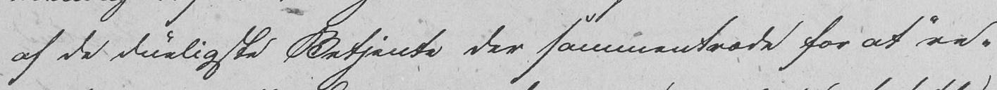af de dueligste Betjente der sammentræde for at "re-
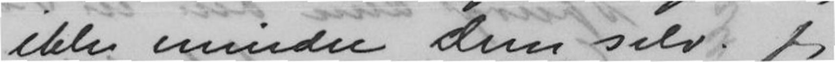ikke mindre Dem selv. Jeg
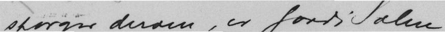spørger derom, er fordi Salen
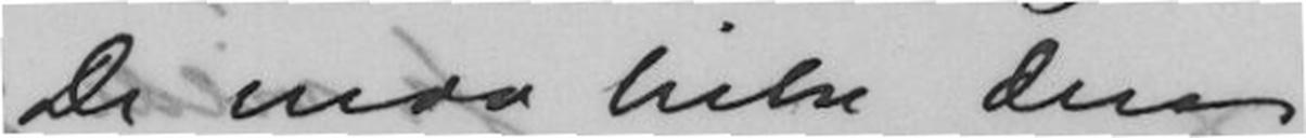De maa hilse Deres
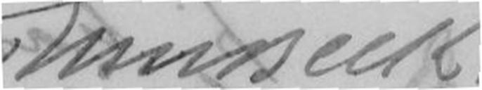Ellen Beck
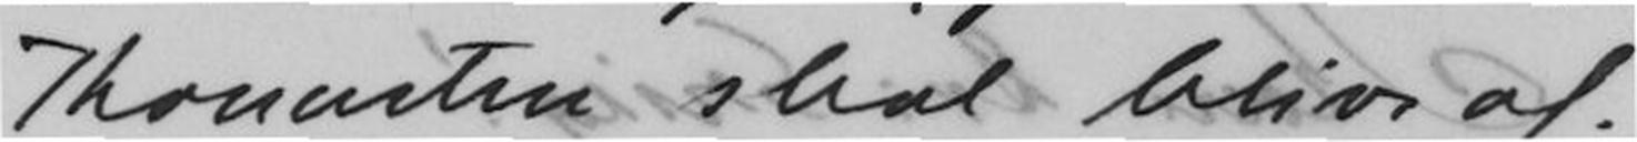Koncerten skal blive af.
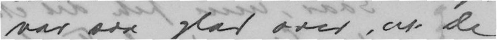var saa glad over, at De
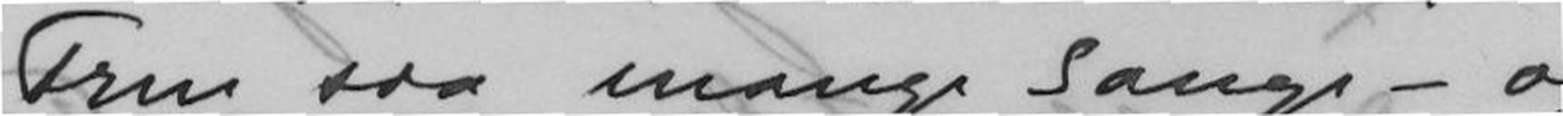Frue saa mange Gange - og
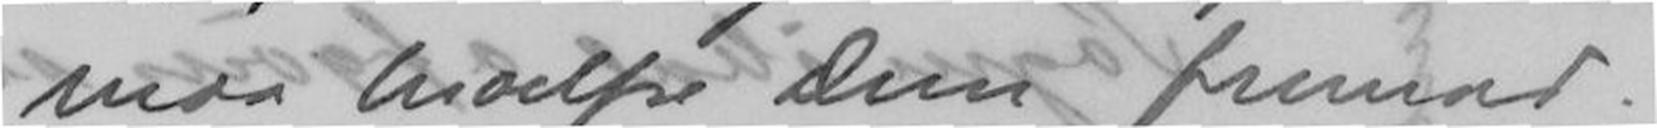maa Mælfri Dem fremad.
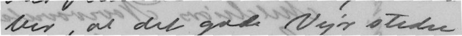ber, at det gode Vejr stedse
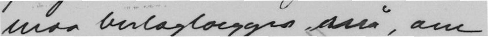maa beslaglægges nu, om
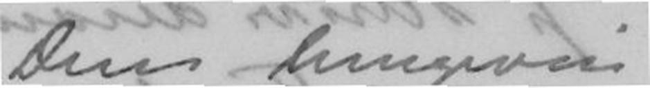Deres hengivne
